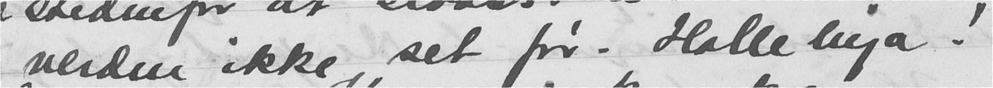verden ikke set før - Halleluja!
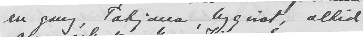en gang, Tatjana, bygvist, altid
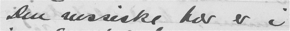Den russiske hær er i
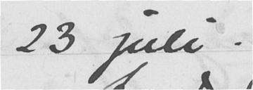23 juli.
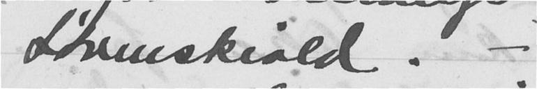Løvenskiold. -
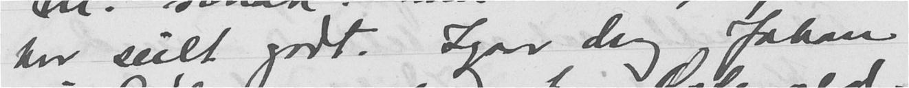har seilt godt. Igaar drog Johan
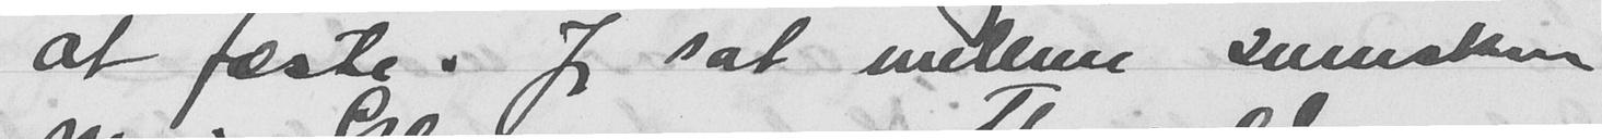at fæste. Jeg sat mellem svensken
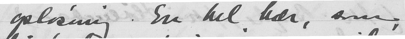opløsning. En hel hær, som
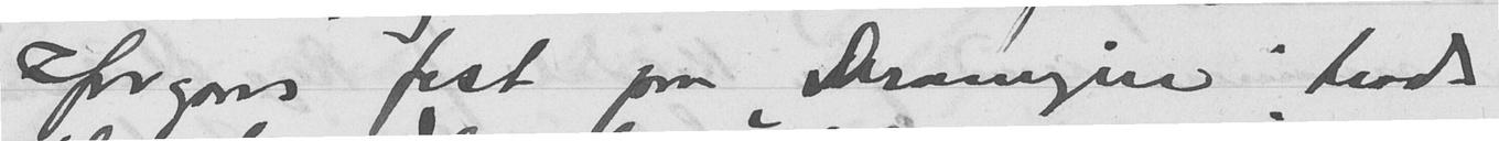Iforgaars fest paa Dronningen trods
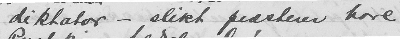diktator - slikt præsterer bare
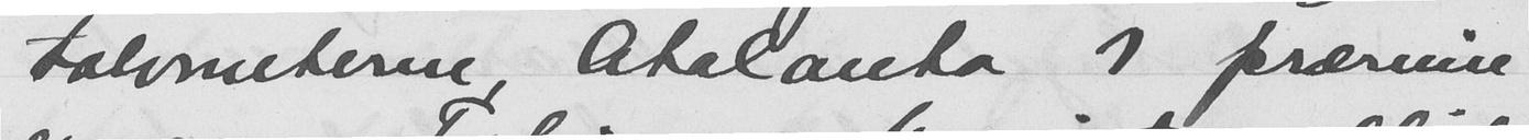tolvmeterne, Atalanta 1 præmie
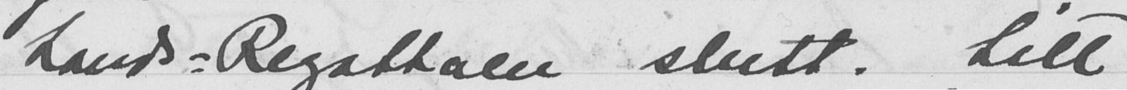Lands-Regattaen slutt. Litt
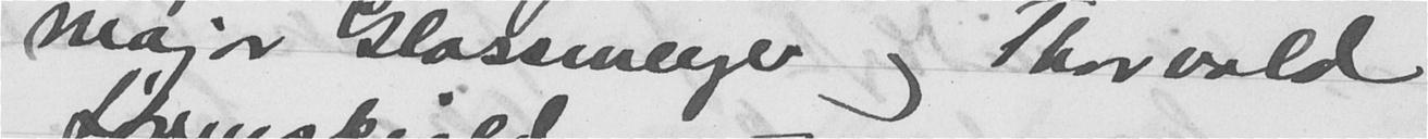major Glassmeyer og Thorvald
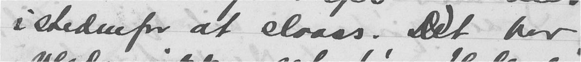istedenfor at slaass. Det har
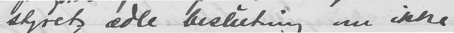styrets ædle beslutning om ikke
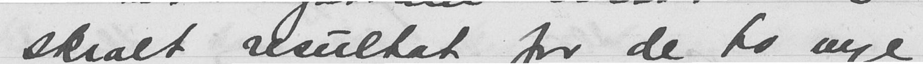skralt resultat for de to nye
